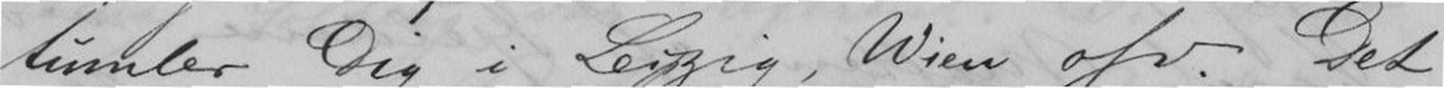tumler Dig i Leipzig, Wien osv. Det
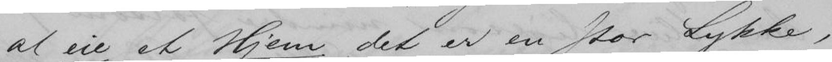at eie et Hjem det er en stor Lykke,
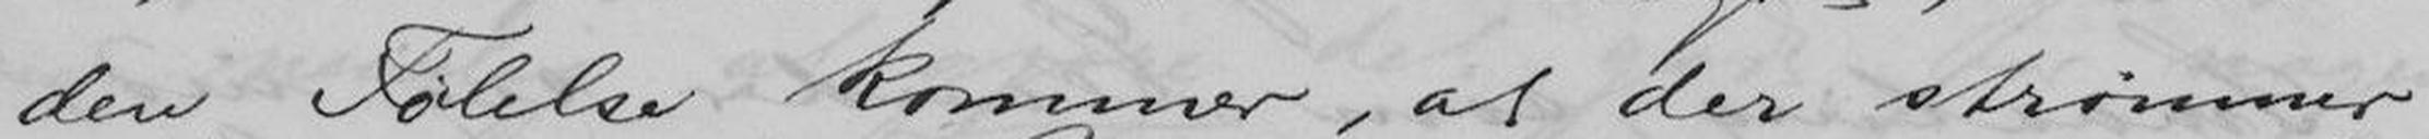den Følelse kommer, at der strømmer
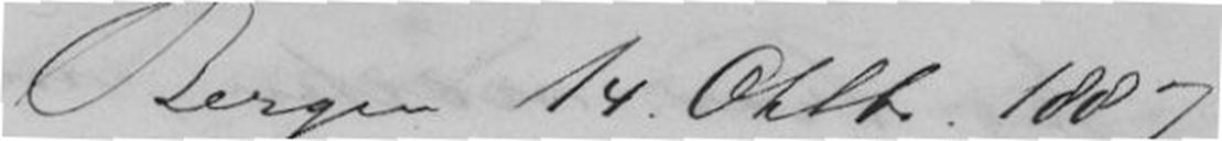Bergen 14 Oktb. 1887.
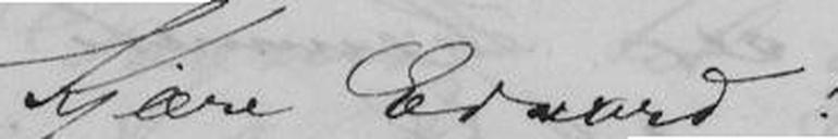Kjære Edvard!
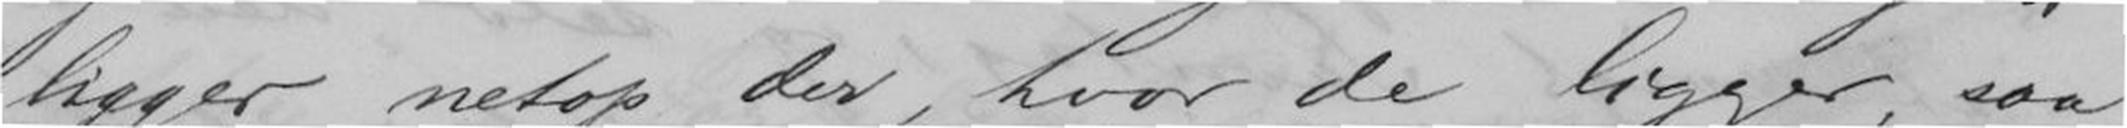ligger netop der, hvor de ligger, saa
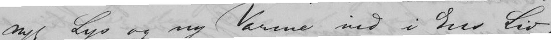nyt Lys og ny Varme ind i Ens Liv,
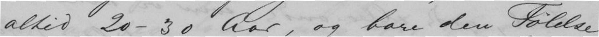altid 20 - 30 Aar, og bare den Følelse
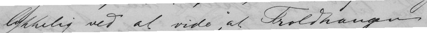kelig ved at vide, at Troldhaugen
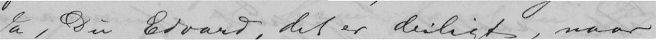Ja, Du Edvard, det er deiligt, naar
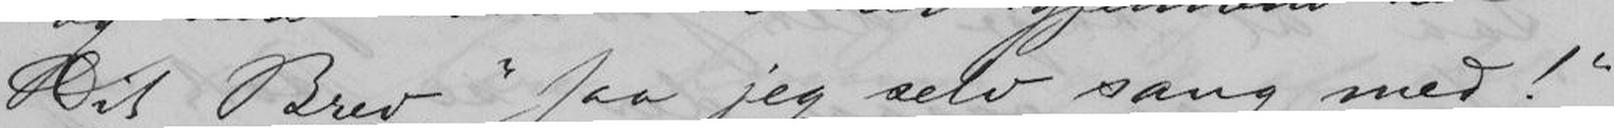Dit Brev, "saa jeg selv sang med!"
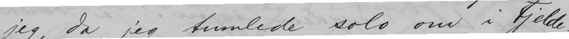jeg, da jeg tumlede solo om i Fjelde-
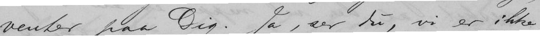venter paa Dig. Ja, ser du, vi er ikke
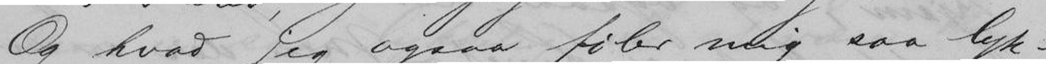Og hvad jeg ogsaa føler mig saa lyk-
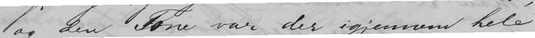og den Tone var der igjennem hele
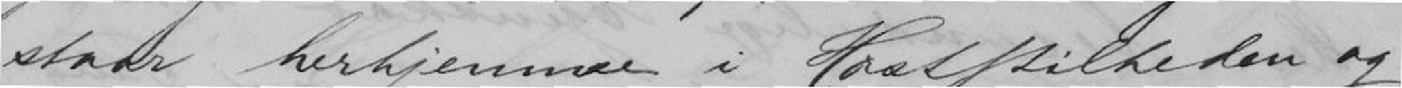staar herhjemme i Høststilheden og
